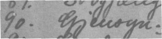90. Gjensyn.
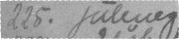225. Juleneg
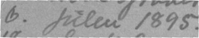6.Julen 1895.
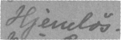Hjemløs.
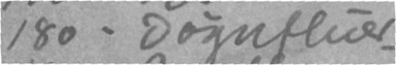180. Døgnfluer
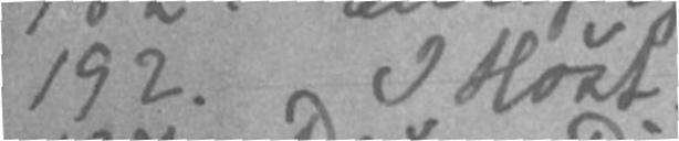192. I Høst.
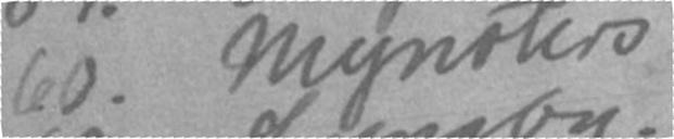60. Mynsters.
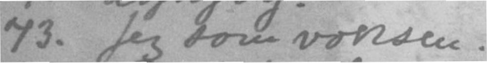73. Jeg som voksen.
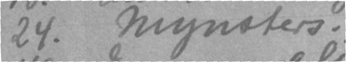24. Mynsters.
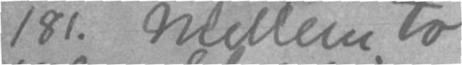181. Mellem to.
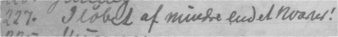227. Iløbet af mindre end et kvarter!
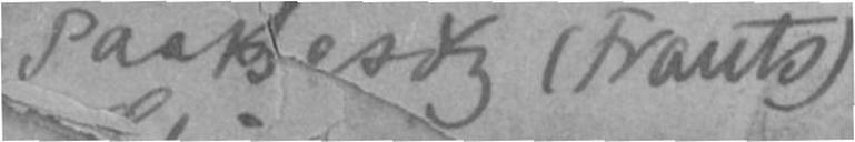Paa besøg (Frants)
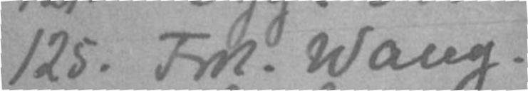125. Frk. Wang.
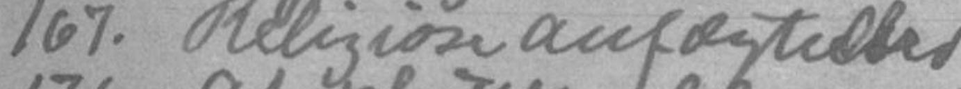167. Religiøse anfægteder
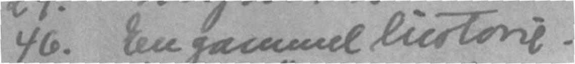46. En gammel historie.
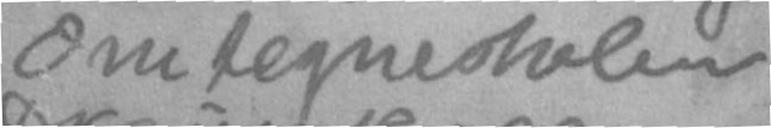255. Om tegneskolen
